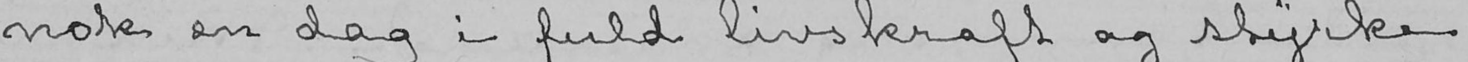nok en dag i fuld livskraft og styrke
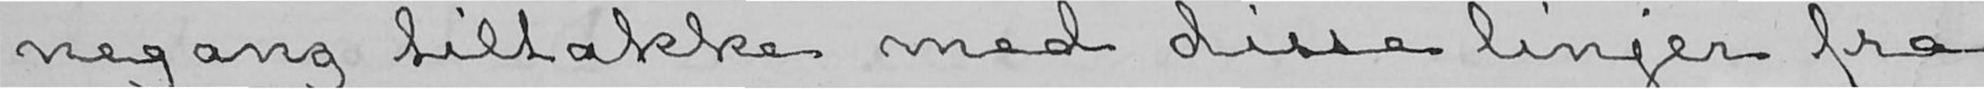negang tiltakke med disse linjer fra
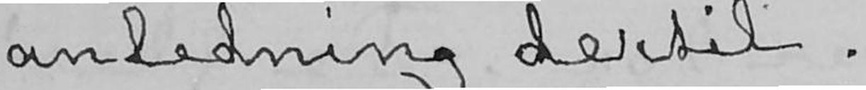anledning dertil.-
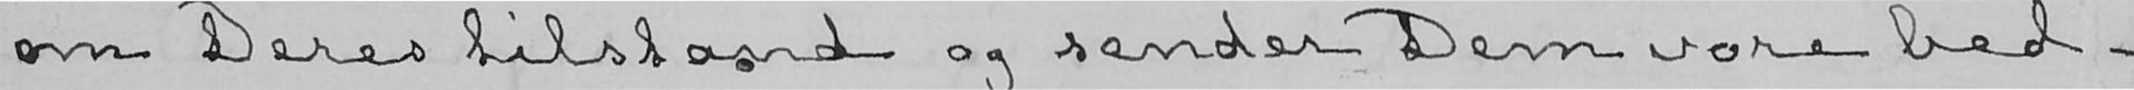om Deres tilstand og sender Dem vore bed-
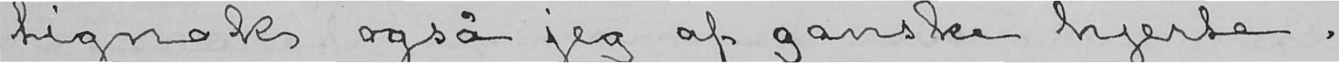tignok også jeg af ganske hjerte.
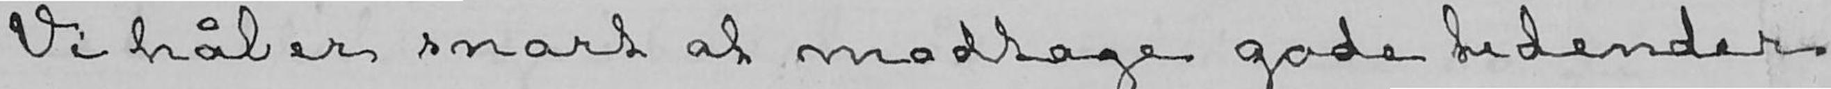Vi håber snart at modtage gode tidender
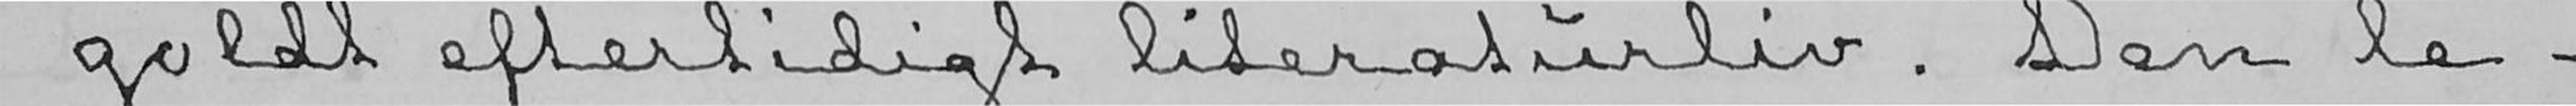goldt eftertidigt literaturliv. Den le-
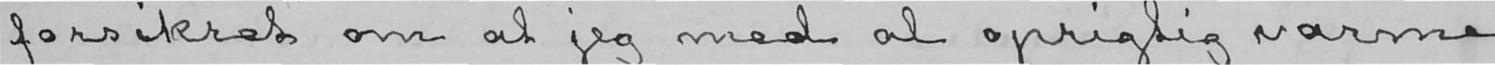forsikret om at jeg med al oprigtig varme
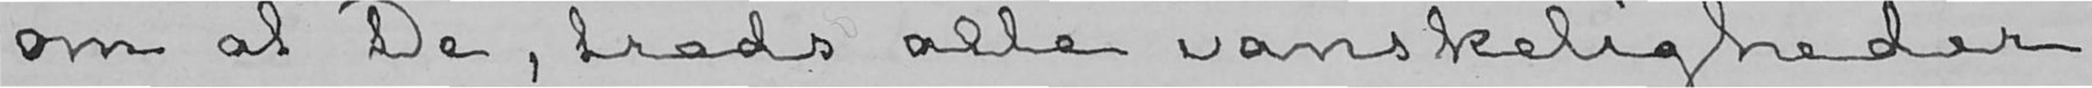om at De, trods alle vanskeligheder
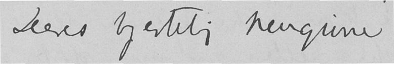Deres hjertelig hengivne
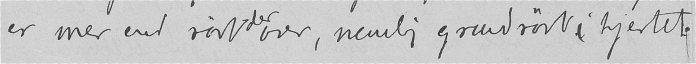er mer end rørt derover, nemlig grundrørt i hjertet.
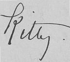Kitty.
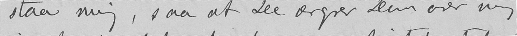staa mig, saa at De ærgrer Dem over mig,
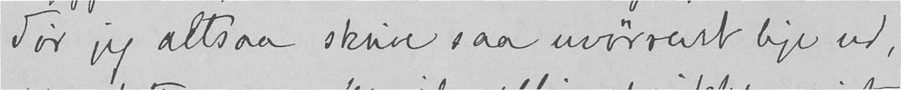Tør jeg altsaa skrive saa uvørrent lige ud,
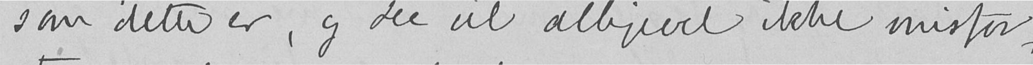som dette er, og De vil alligevel ikke misfor-
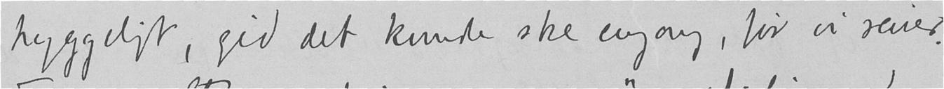hyggeligt, gid det kunde ske engang, før vi reiser.
